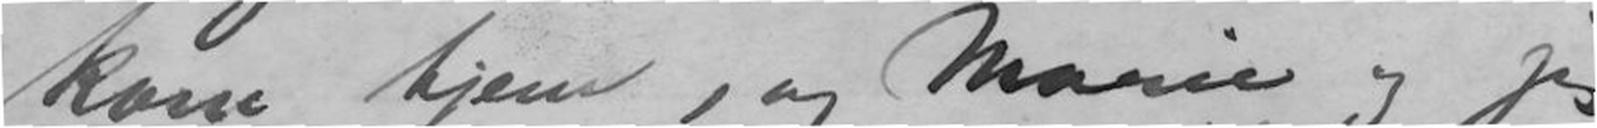kom hjem, og Marie og jeg
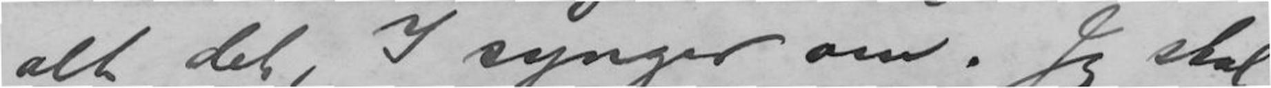alt det, I synger om. Jeg skal
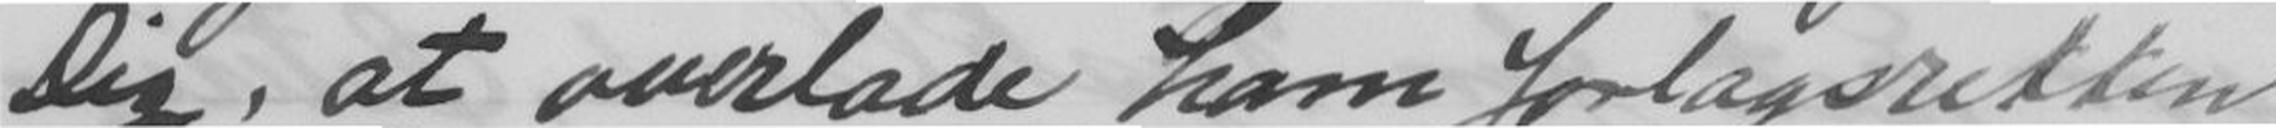Dig, at overlade ham forlagsretten
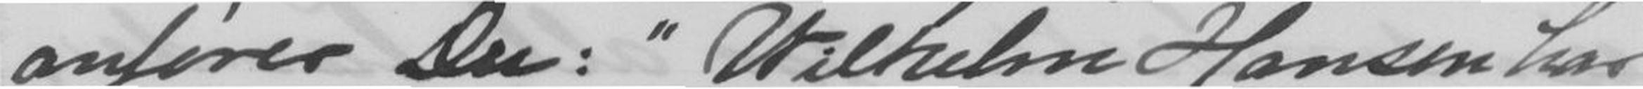anfører Du: "Wilhelm Hansen har
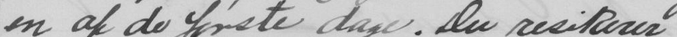en af de første dage. Du resikerer
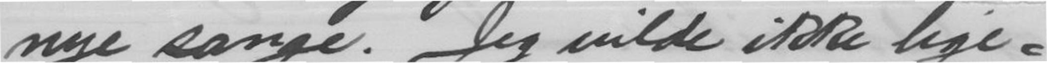nye sange. Jeg vilde ikke lige.
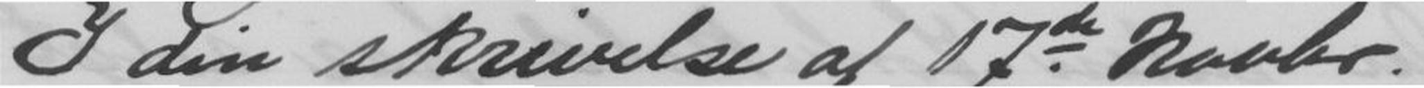I din skrivelse af 17de Novbr.
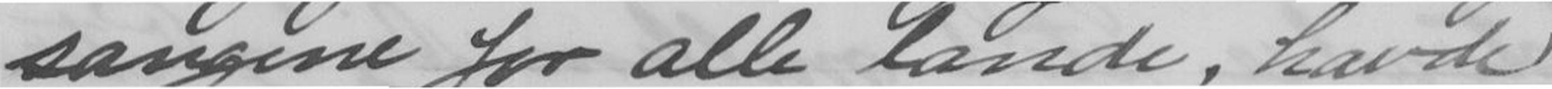sangene for alle lande, havde
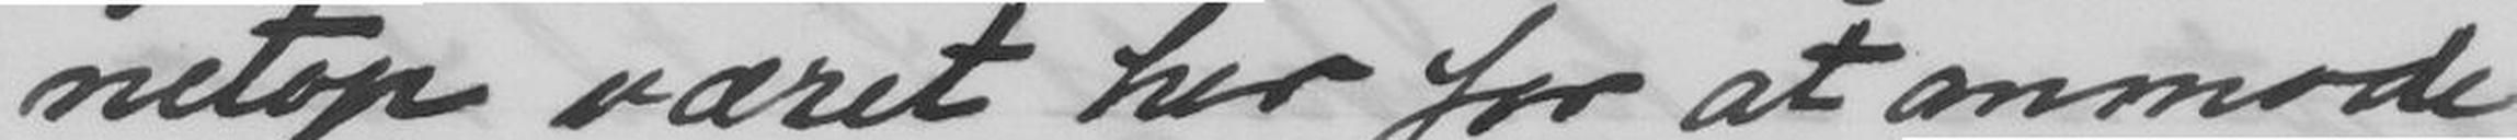netop været her for at enmode
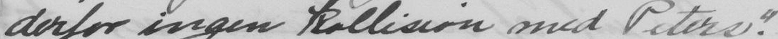derfor ingen kollision med Peters".
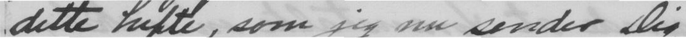dette hefte, som jeg nu sender Dig
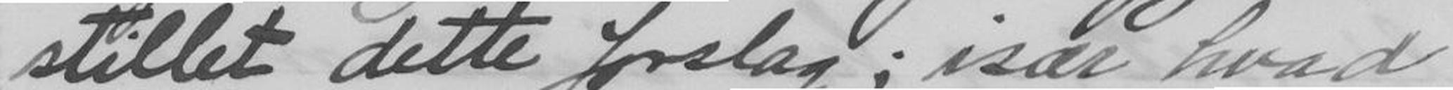stillet dette forslag; især hvad
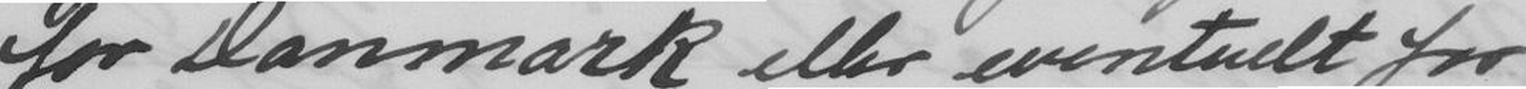for Danmark eller eventuelt for
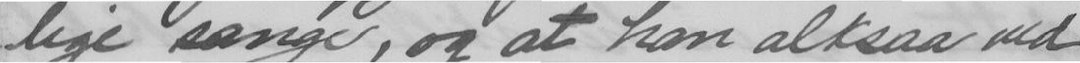lige sange, og at han altsaa ved
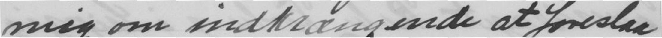mig om indtrængende at foreslaa
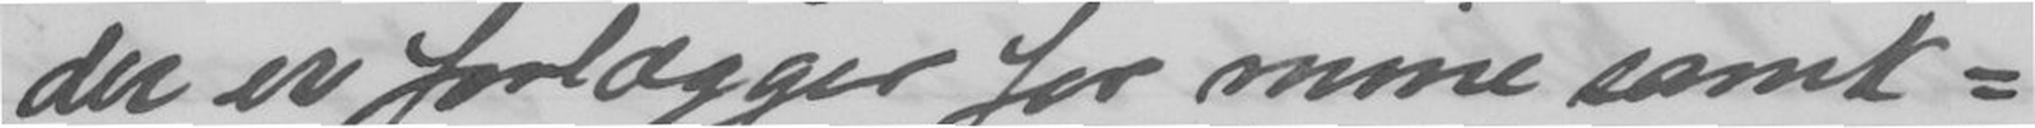der er forlægger for mine samt=
